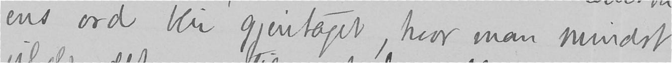ens ord blir gjentaget, hvor man mindst
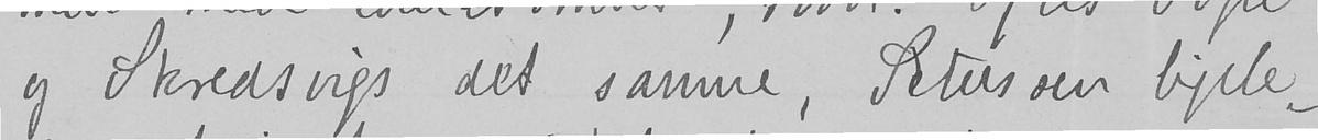og Skredsvigs det samme, Peterssen ligele-
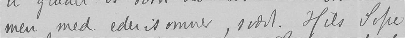men med eder isommer, svært. Hils Sofie
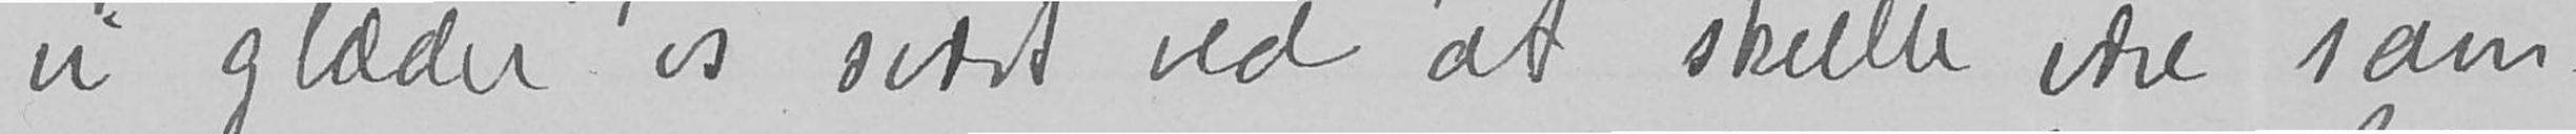vi glæder os svært ved at skulle være sam-
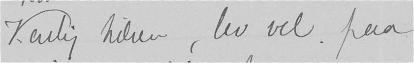Venlig hilsen, lev vel, paa
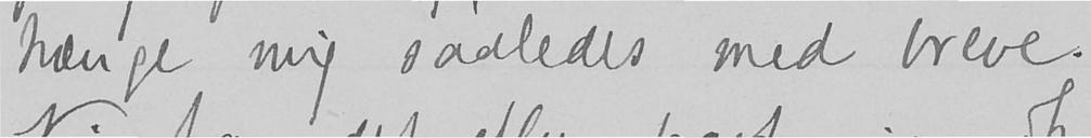hænge mig saaledes med breve.
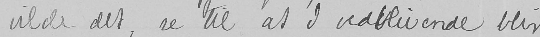vilde det, se til at I vedblivende blir
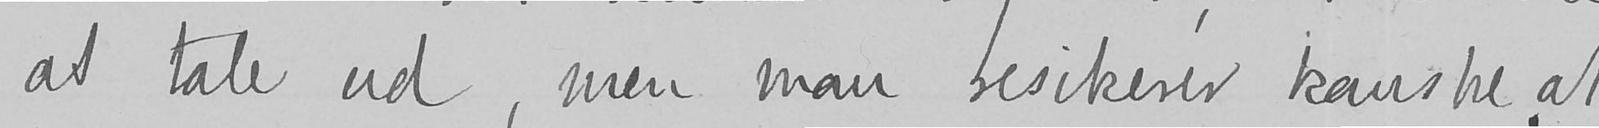at tale ud, men man resikerer kanske, at
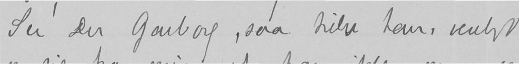Ser Du Garborg, saa hilse han venligt
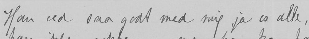Han ved saa godt med mig, ja os alle,
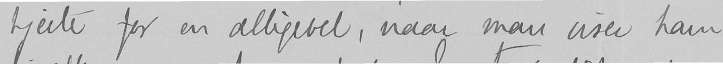hjerte for en alligevel, naar man viser ham
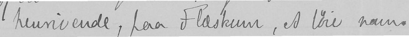henrivende, paa Flæskum, et løie navn.
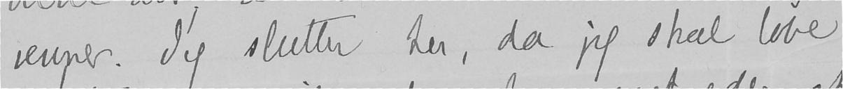venner. Jeg slutter her, da jeg skal løbe
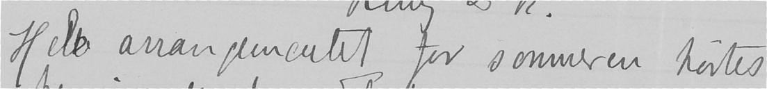Hele arrangementet for sommeren hørtes
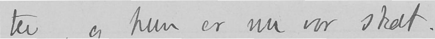ter, og hun er nu vor skat.
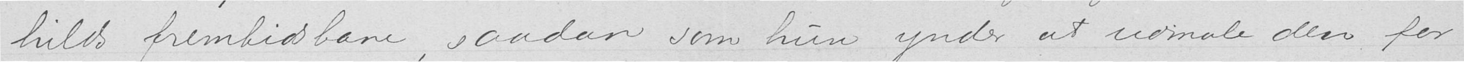hilds fremtidsbane, saadan som hun ynder at udmale den for
